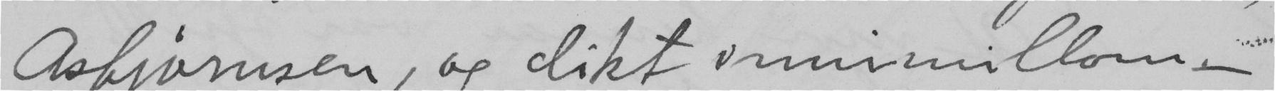Asbjörnsen, og dikt innimillom.
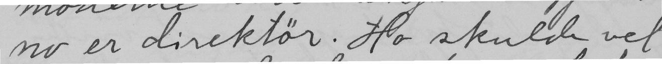no er direktör. Ho skulde vel
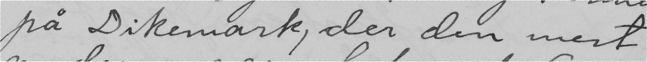på Dikemark, der den mest
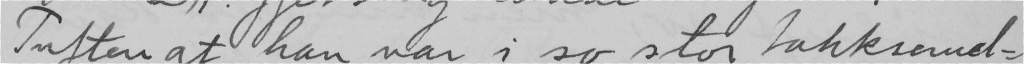Tuften at han var i so stor takksemd-
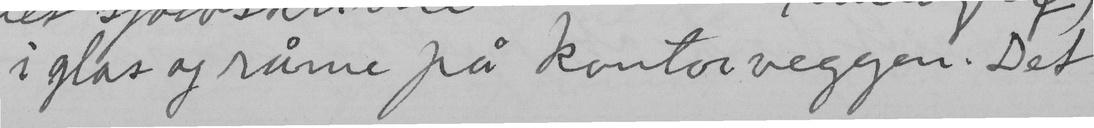i glas og råme på kontorveggen. Det
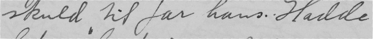skuld til far hans. Hadde
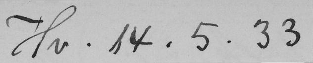Hv. 14.5.33
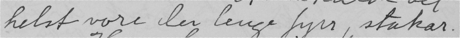helst vore der lenge fyrr, stakar.
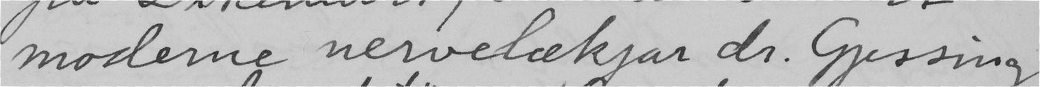moderne nervelækjar dr. Gjessing
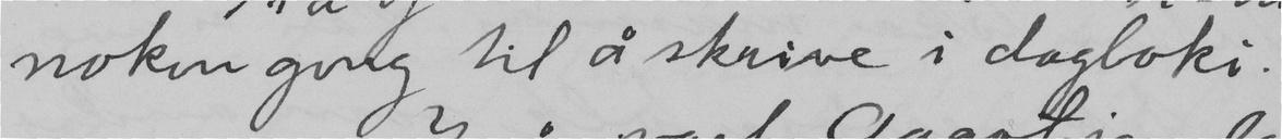nokon gong til å skrive i dagboki.
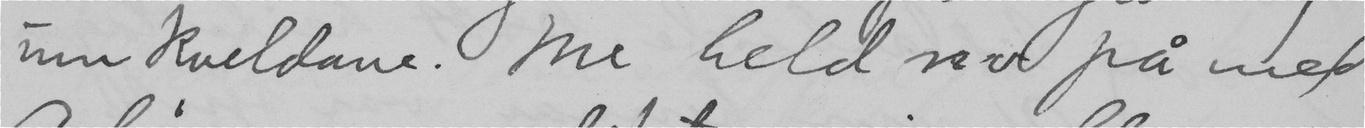um kveldane. Me held no på med
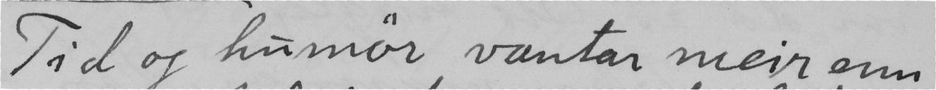Tid og humör vantar meir enn
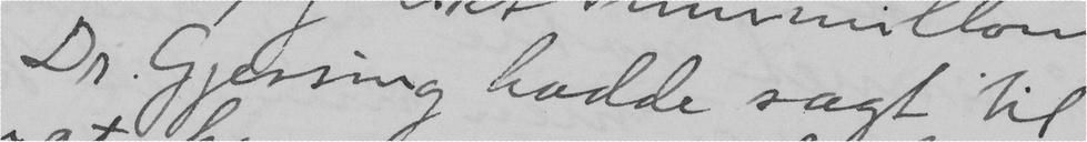Dr. Gjessing hadde sagt til
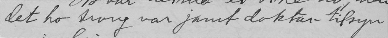det ho trong var jamt doktar-tilsyn
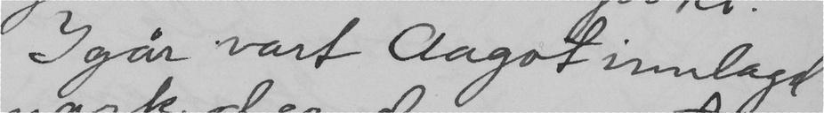Igår vart Aagot innlagt
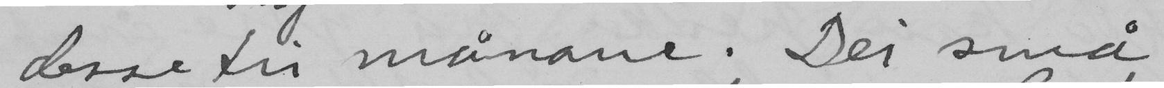desse tri månane. Dei små
